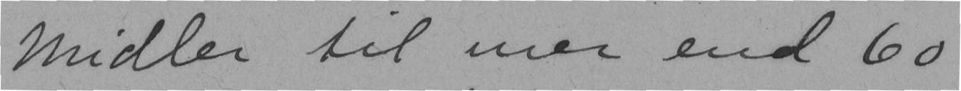midler til mer end 60
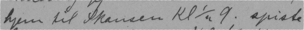hjem til Skansen Kl ½ 9. spiste
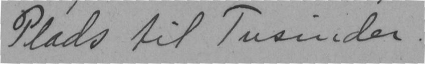Plads til Tusinder.
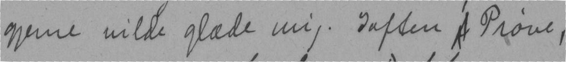gjerne vilde glæde mig. Iaften  Prøve,
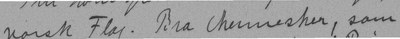norsk Flag. Bra Mennesker, som
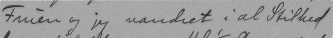Fruen og jeg vandret i al Stilhed
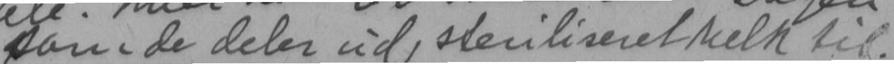som de deler ud, steriliseret melk til.
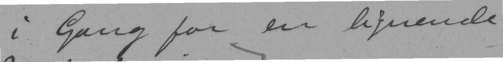i Gang for en lignende
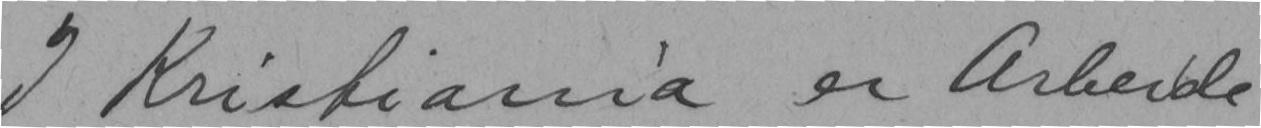I Kristiania er Arbeide
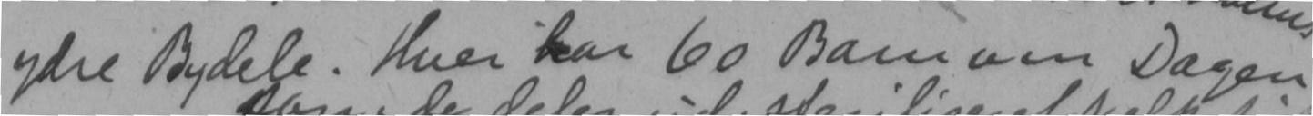ydre Bydele. Hver har 60 Barn om Dagen
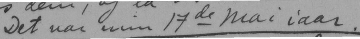Det var min 17de Mai iaar.
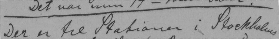Der er tre Stationer i Stockholms
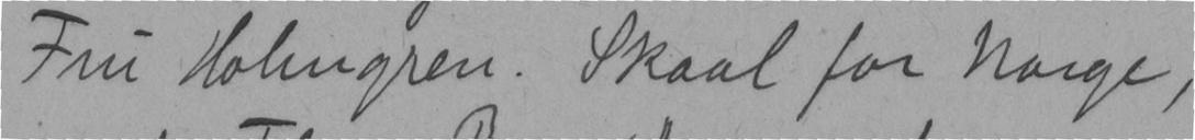Fru Holmgren. Skaal for Norge,
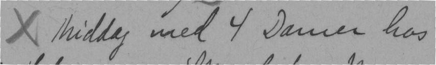x Middag med 4 Damer hos
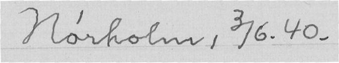Nørholm, 3/6. 40.
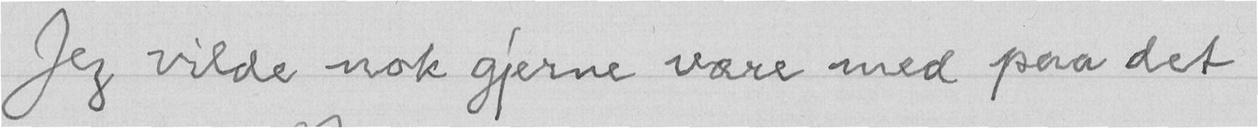Jeg vilde nok gjerne være med paa det
